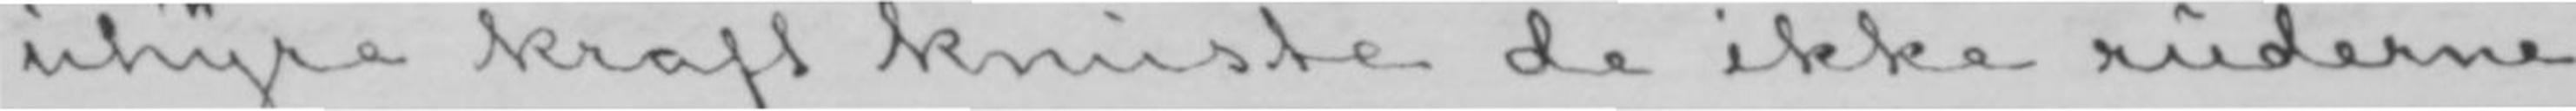uhyre kraft knuste de ikke ruderne
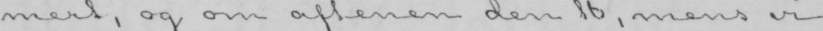mert, og om aftenen den 16, mens vi
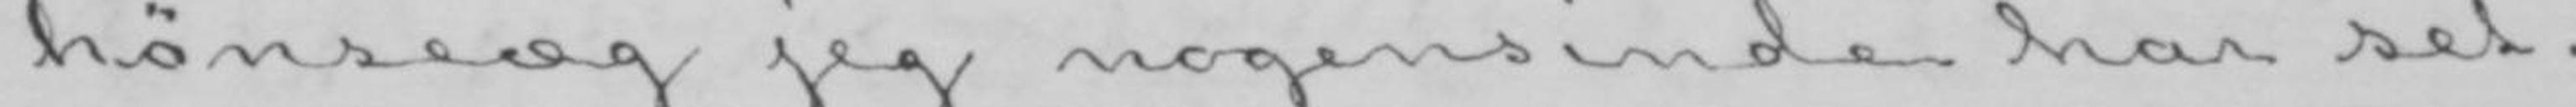hønseæg jeg nogensinde har set;
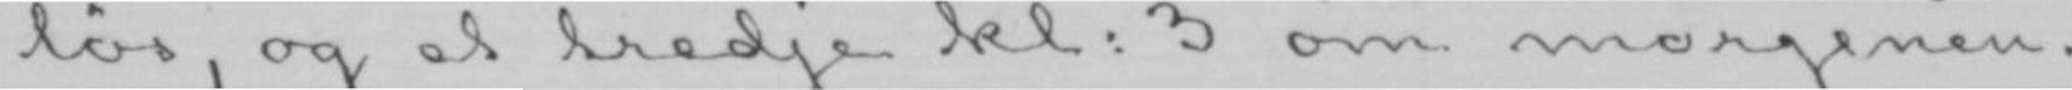løs, og et tredje kl: 3 om morgenen.
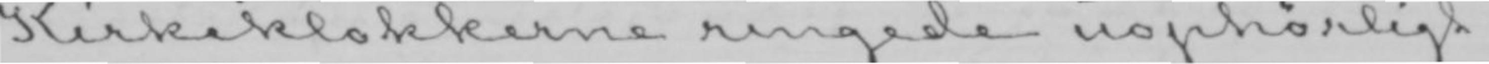Kirkeklokkerne ringede uophørligt,
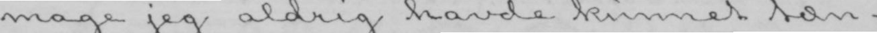mage jeg aldrig havde kunnet tæn-
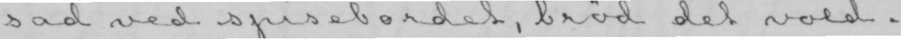sad ved spisebordet, brød det vold-
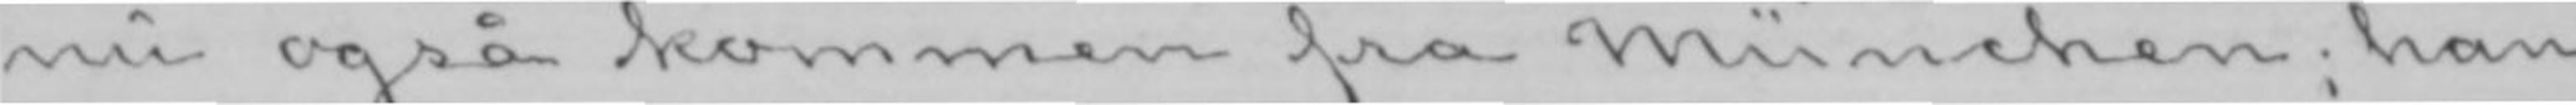nu også kommen fra München; han
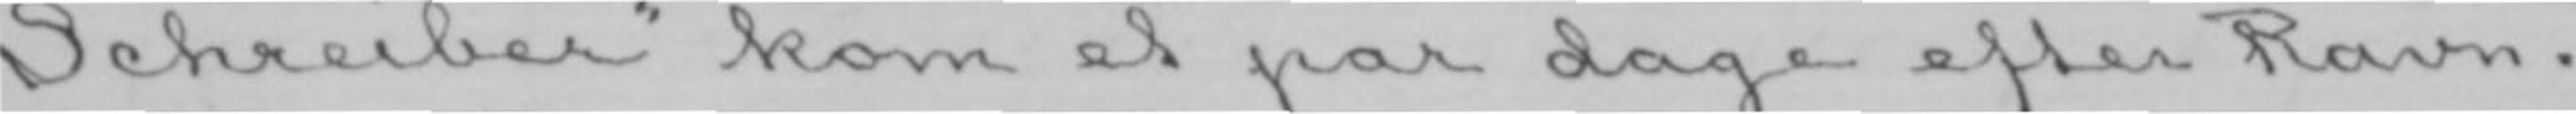Schreiber» kom et par dage efter Ravn-
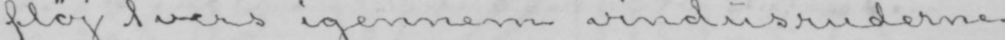fløj tvers igennem vindusruderne
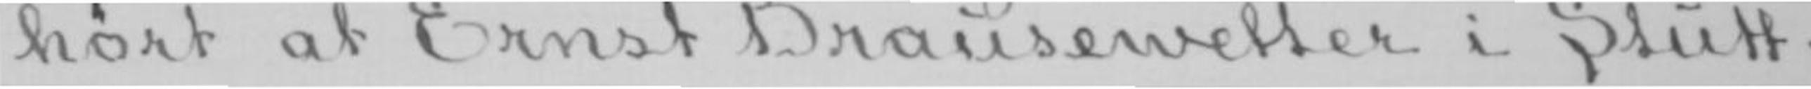hørt at Ernst Brausewetter i Stutt-
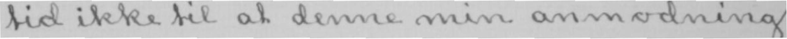tid ikke til at denne min anmodning
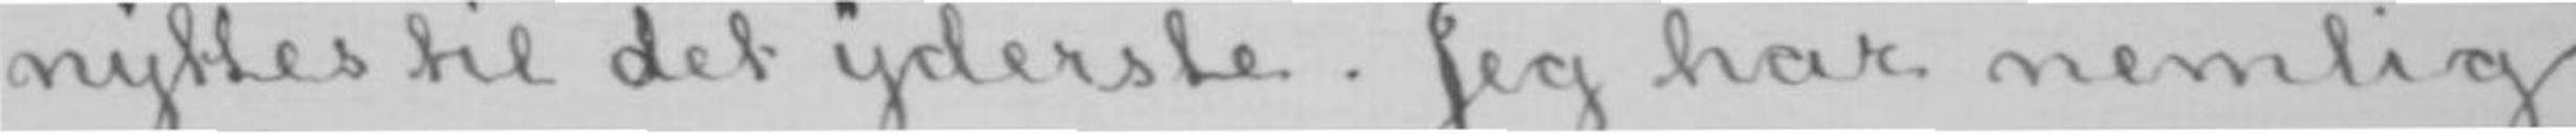nyttes til det yderste. Jeg har nemlig
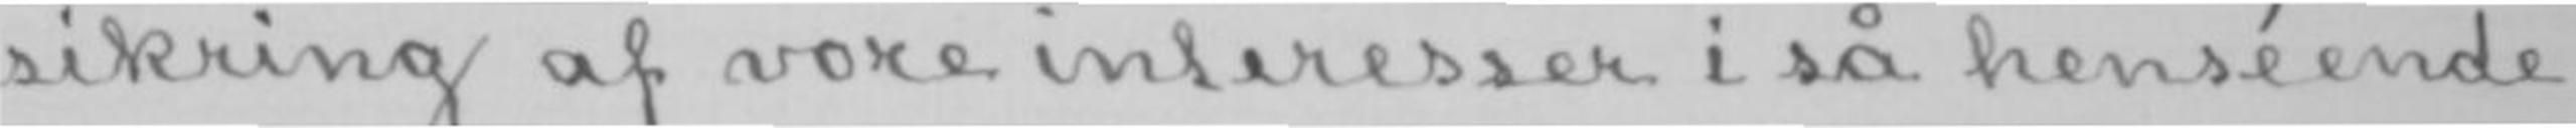sikring af vore interesser i så henséende
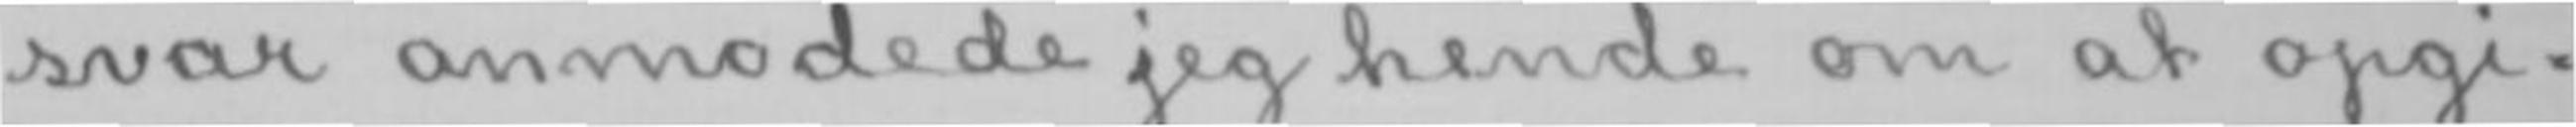svar anmodede jeg hende om at opgi-
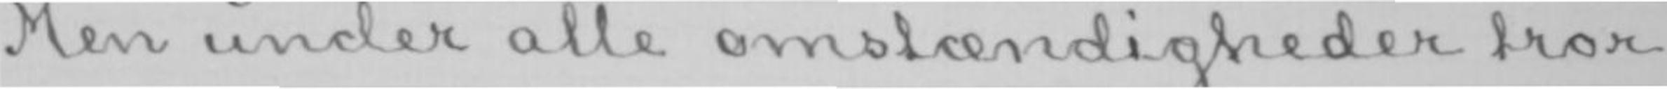Men under alle omstændigheder tror
